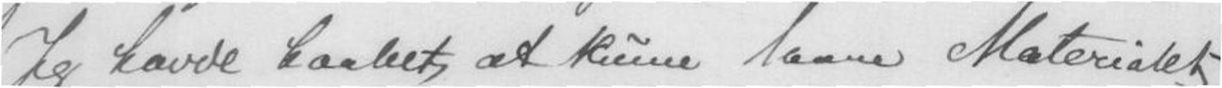Jeg havde haabet at kunne laane Materialet
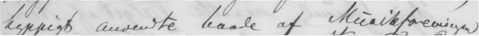hyppigt anvendte baade af Musikforeningen
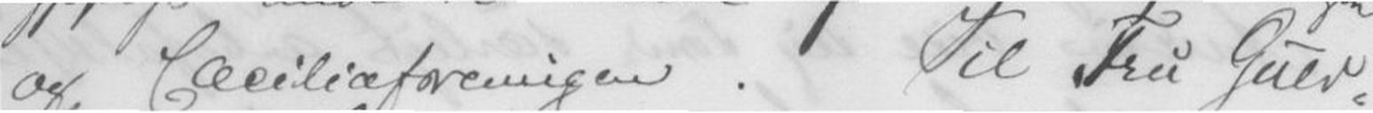og Cæciliæforeningen.Til Fru Gulv-
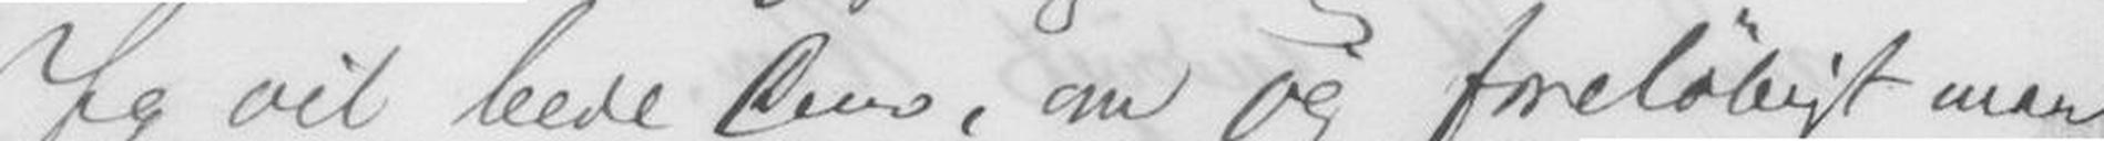Jeg vil bede dem, om og foreløbigt
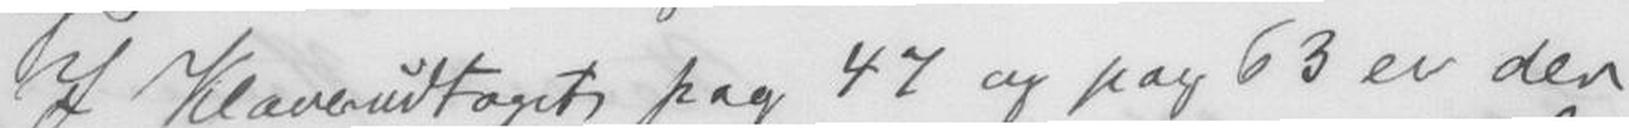I Klaverudtagets pag 47 og pag 63 er den
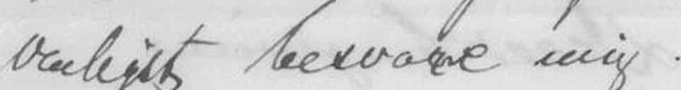vænligst besvare mig.
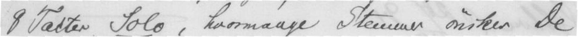8 Takter Solo, hvormange Stemmer ønsker De
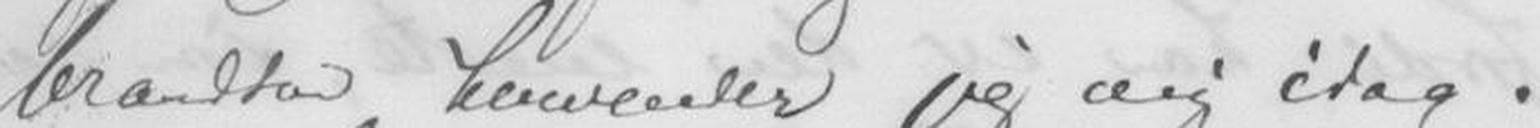brandtsen henvender jeg mig idag.
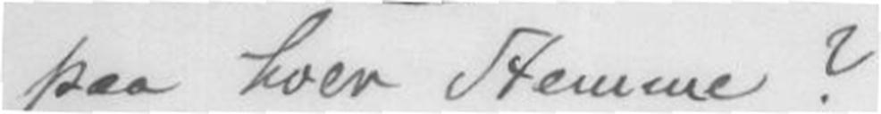paa hver Stemme?
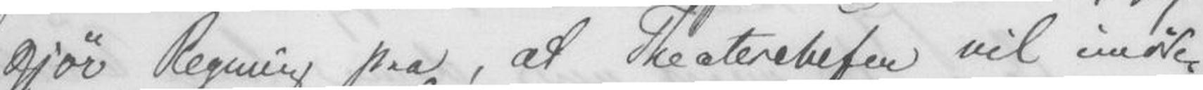gjør Regning paa, at Theaterchefen vil ind-
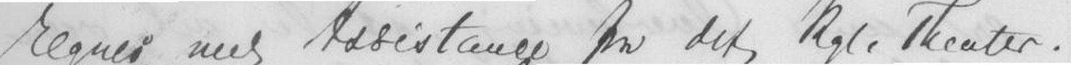regnes med Assistance fra det Kgl. Theater.
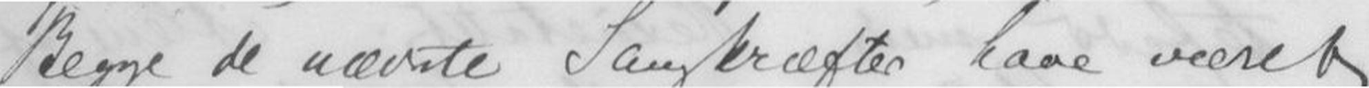Begge de nævnte Sangkræfter have været
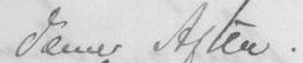denne Aften.
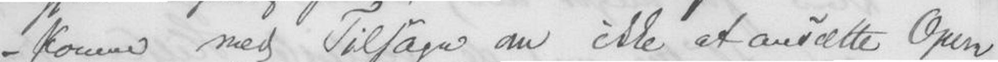komme med Tilsang om ikke at ansætte Open
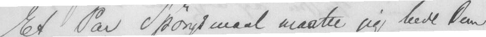Et Par Spørgsmaal maatte jeg bede Dem
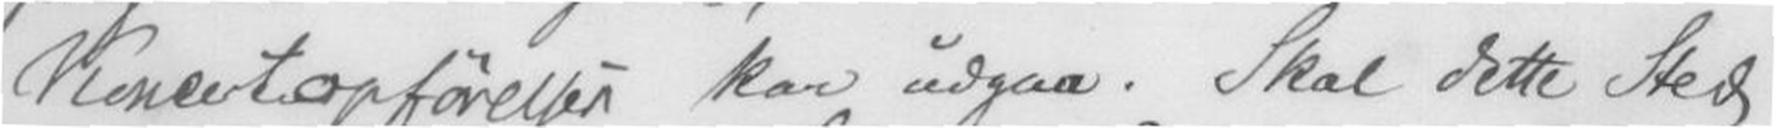Koncertopførelsen kan udgaa. Skal dette Sted
